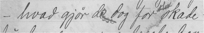- hvad gjør de dog for skade!
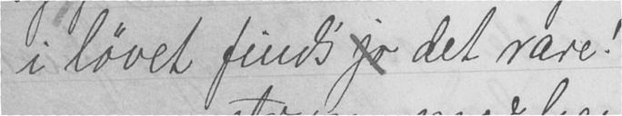i løvet finds jo det rare!
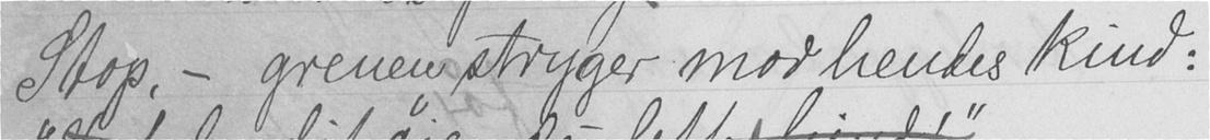Stop, - grenen stryger mod hendes kind:
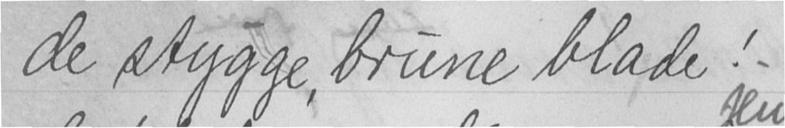de stygge, brune blade!
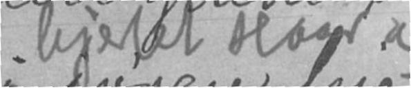hjertet haar
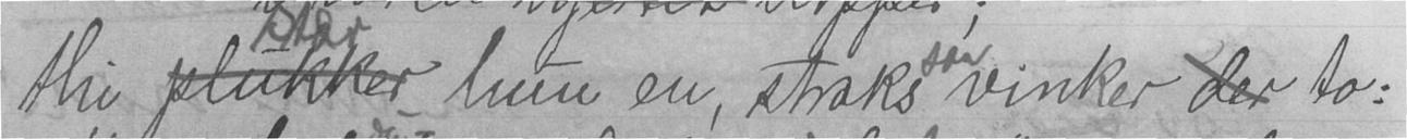thi star hun en, straks saa vinker der to:
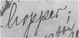hopper;
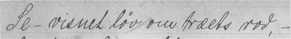Se - visnet løv om træets rod, -
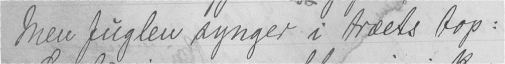Men fuglen synger i træets top:
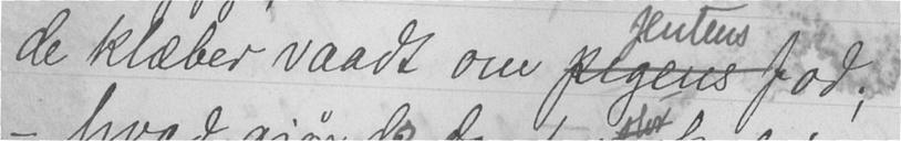de klæber vaadt om jentens fod;
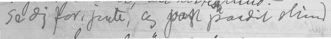Se dig for jente, og pass paadit skind)
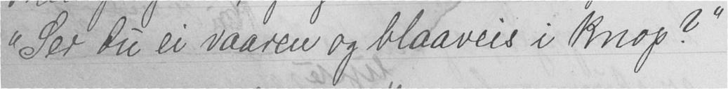"Ser du ei vaaren og blaaveis i knop?"
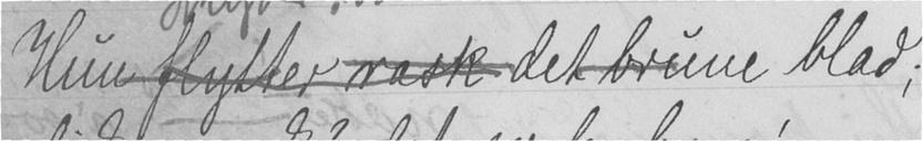Hun skyver væk det brune blad;
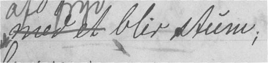af fryd blir stum;
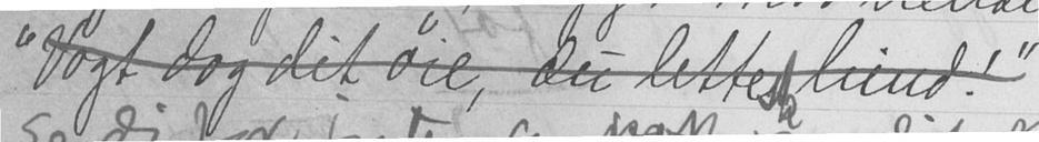"Vogt dog dit øie, du letteste hind!"
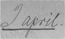I april.
